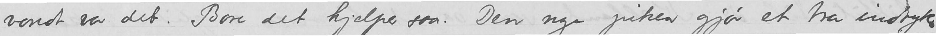vondt var det. Bare det hjelper saa. Den nye piken gjør et bra indtryk
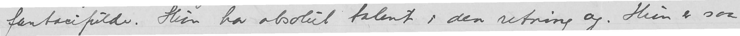og fantacifulde. Hun har absolut talent i den retning og. Hun er
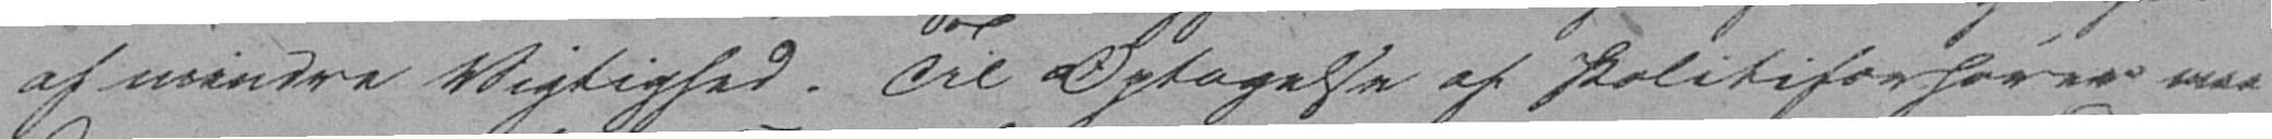af mindre Vigtighed. Til Optagelse af Politiforhører maa
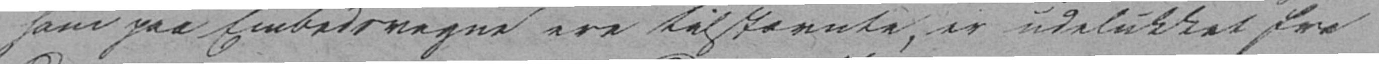ham paa Embedsvegne ere tilstævnte, er udelukket fra
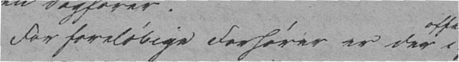For foreløbige Forhører er der i
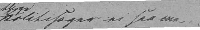Politisager ei saa me-
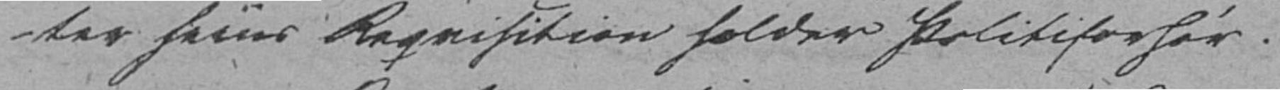ter hans Reqvisition holder Politiforhør.
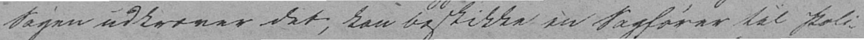Sagen udkræver det, kan beskikke en Sagfører til Poli-
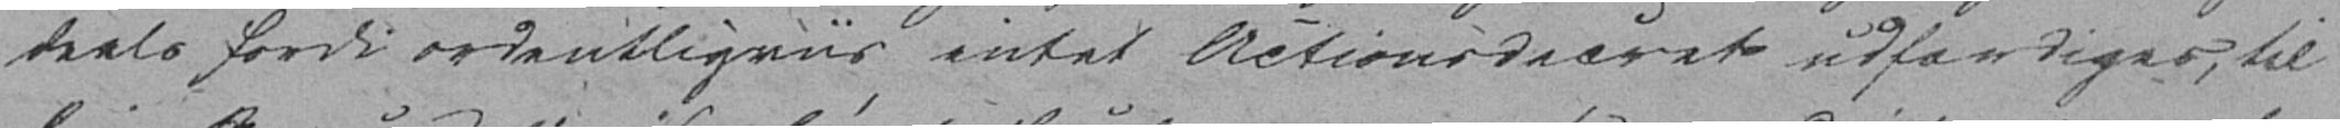deels fordi ordentligviis intet Actionsdecret udfærdiges, til
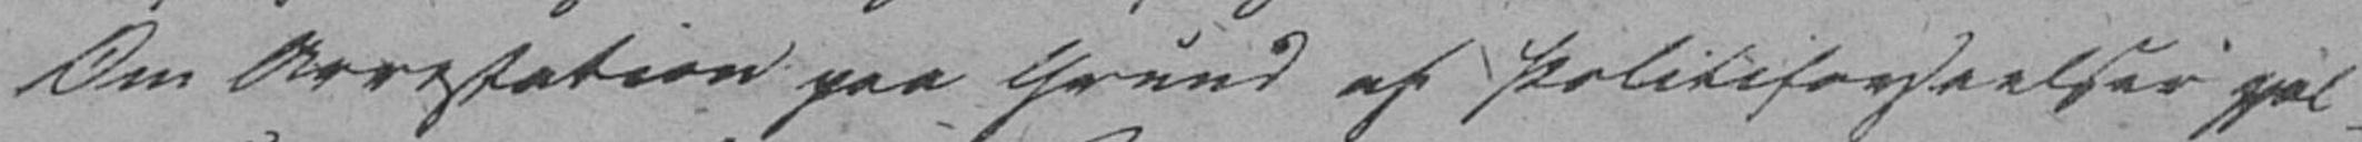Om Arrestation paa Grund af Politiforseelser gjel-
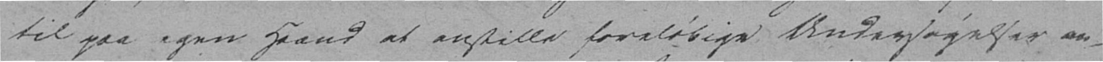til paa egen Haand at anstille foreløbige Undersøgelser an-
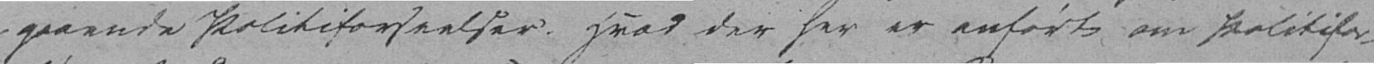gaaende Politiforseelser. Hvad der her er anført om Politifor-
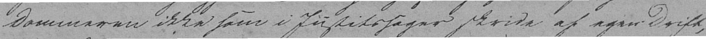Dommeren ikke som i Justitssager skride af egen Drift,
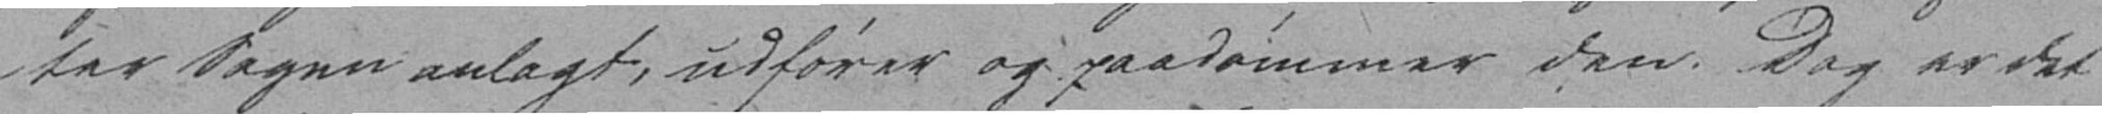ter Sagen anlagt, udfører og paadømmer den. Dog er det
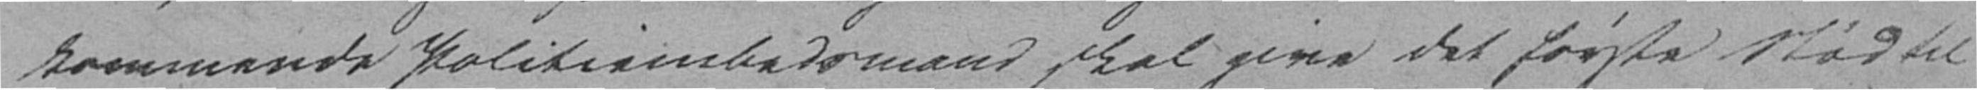kommende Politiembedsmand skal give det første Nød til
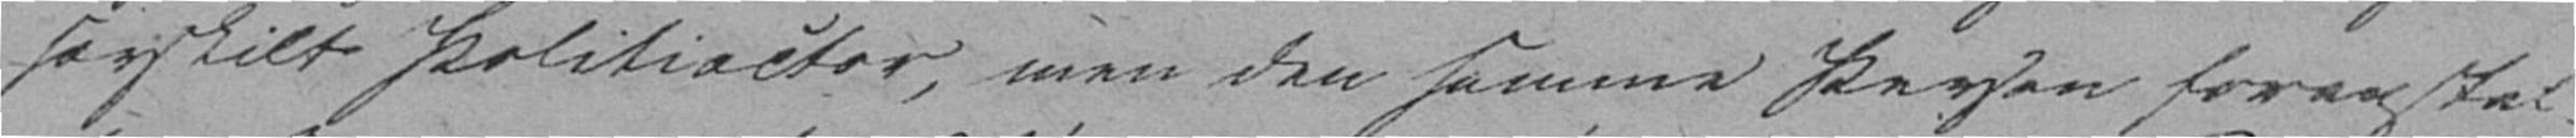særskilt Politiactor, men den samme Person foranstal-
